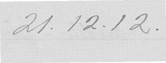21.12.12.
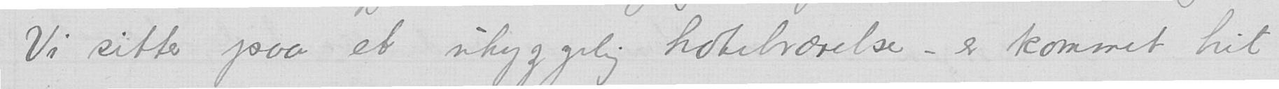Vi sitter paa et uhyggelig hotelværelse - er kommet hit
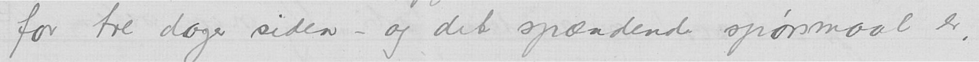for tre dager siden - og det spændende spørsmaal er,
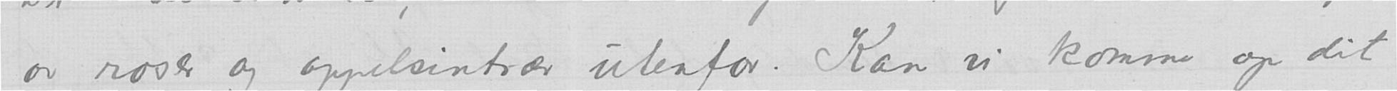av roser og appelsintrær utenfor. Kan vi komme op dit
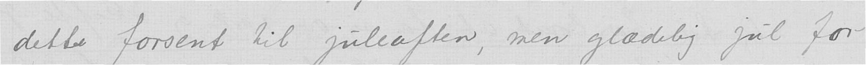dette forsent til juleaften, men glædelig jul for
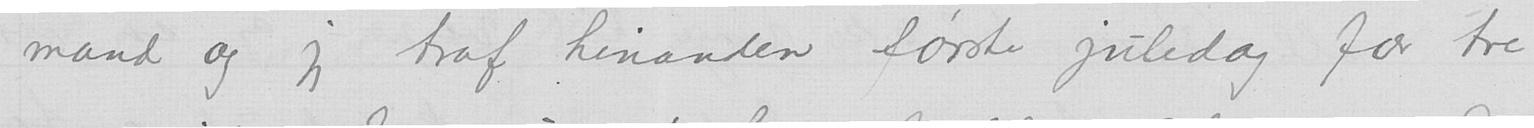mand og jeg traf hinanden første juledag for tre
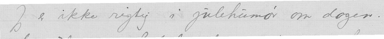Jeg er ikke rigtig i julehumør om dagen.
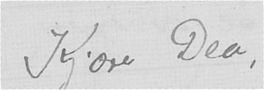Kjære Dea,
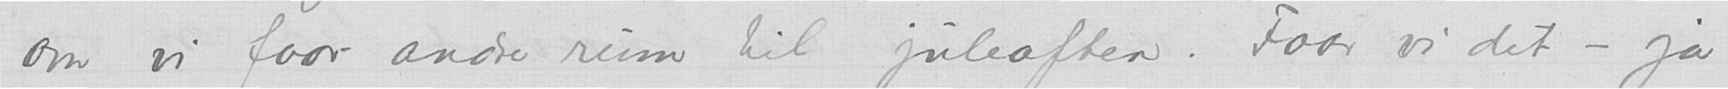om vi faar andre rum til juleaften. Faar vi det - ja
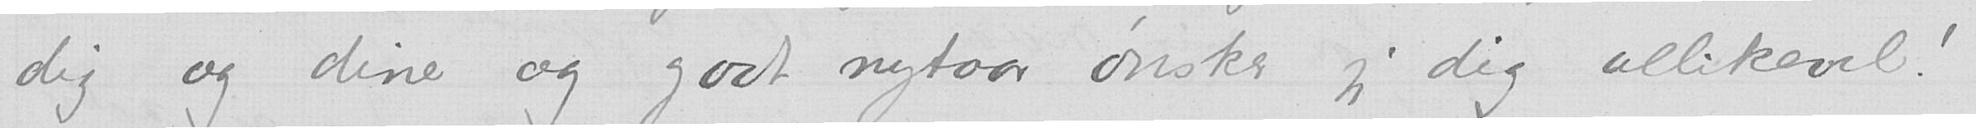dig og dine og godt nytaar ønsker jeg dig allikevel!
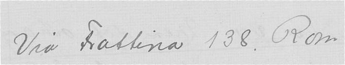Via Frattina 138. Rom
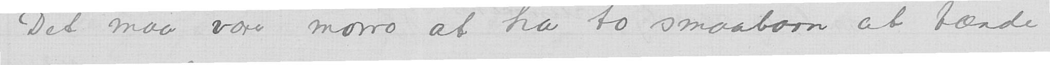Det maa være morro at ha to smaabarn at tænde
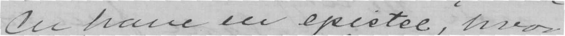du have en epistel, hvor
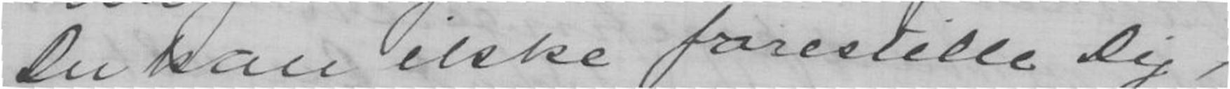Du kan ikke forestille Dig,
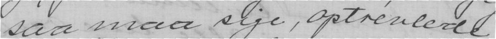saa maa sige, optrevlede
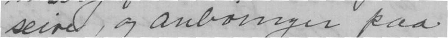seire, og anbringer paa
